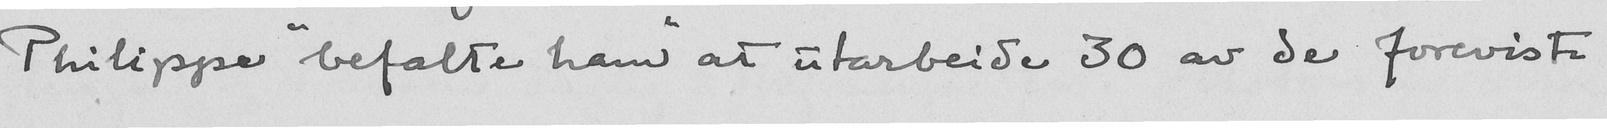Philippe "befalte ham" at utarbeide 30 av de foreviste
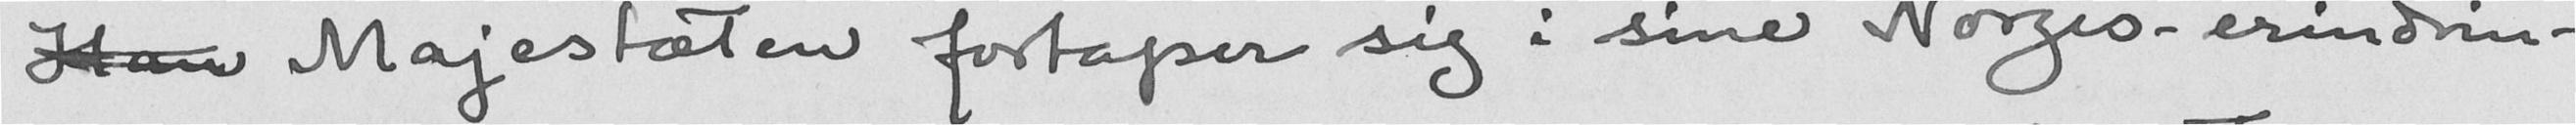Han Majestæten fortaper sig i sine Norges-erindrin-
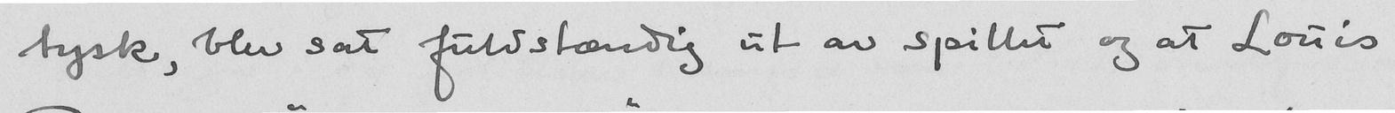tysk, blev sat fuldstændig ut av spillet og at Louis
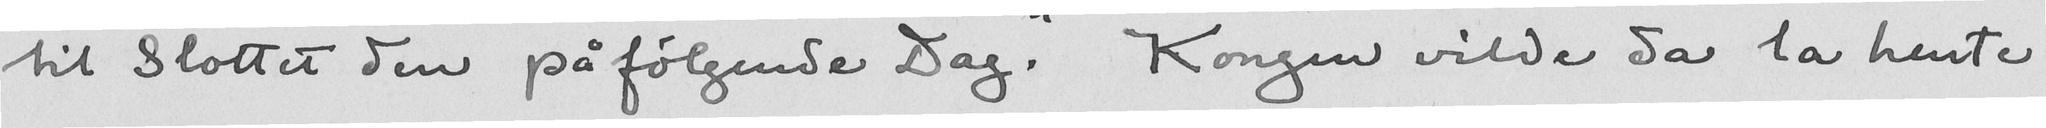til Slottet den påfølgende Dag." Kongen vilde da la hente
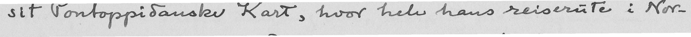sit Pontoppidanske Kart, hvor hele hans reiserute i Nor-
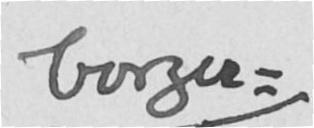borger-
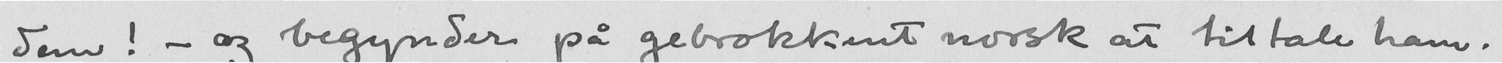dem! - og begynder på gebrokkent norsk at tiltale ham.
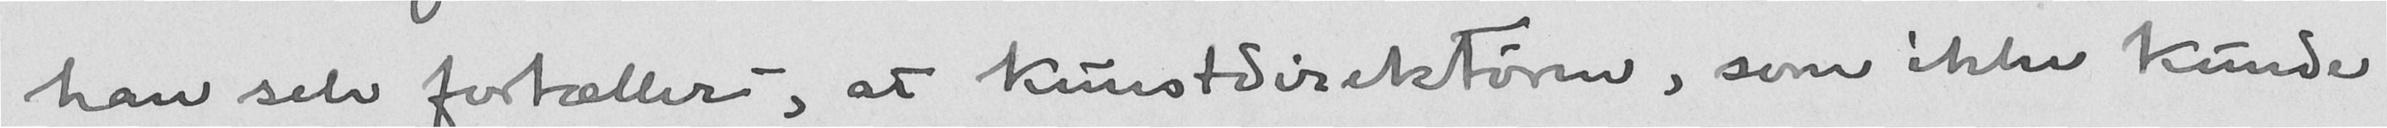han selv fortæller, at kunstdirektøren, som ikke kunde
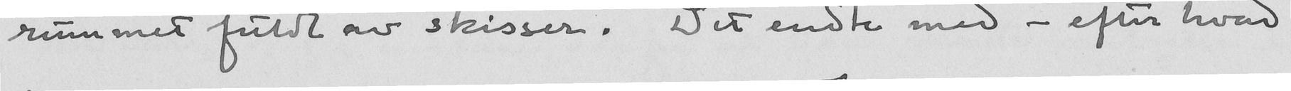rummet fuldt av skisser. Det endte med - efter hvad
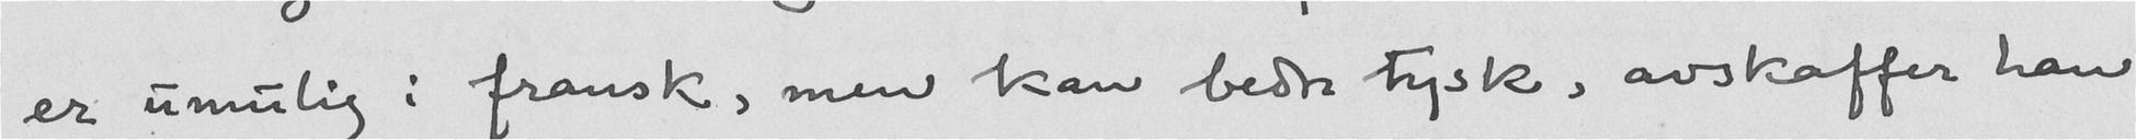er umulig i fransk, men kan bedre tysk, avskaffer han
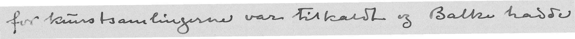for kunstsamlingerne var tilkaldt og Balke hadde
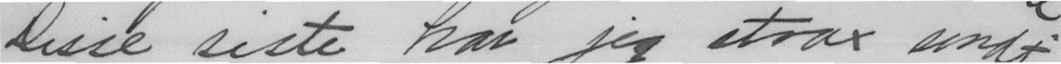Disse siste har jeg strax sendt
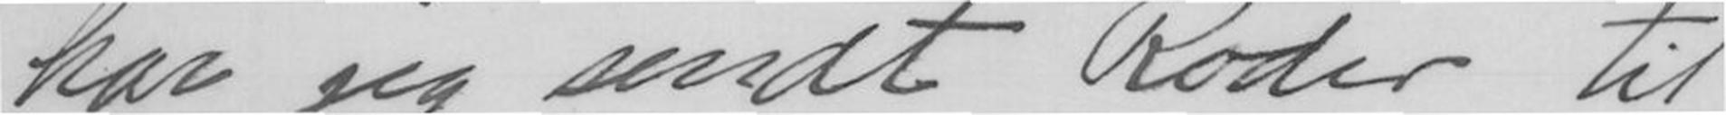har jeg sendt Röder til
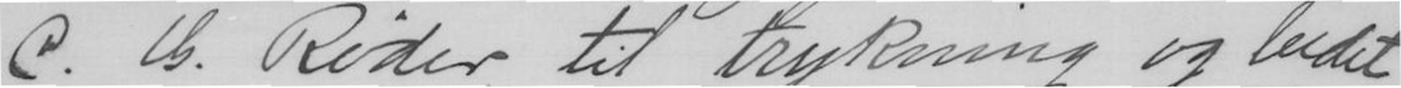C. G. Röder til trykning og bedet
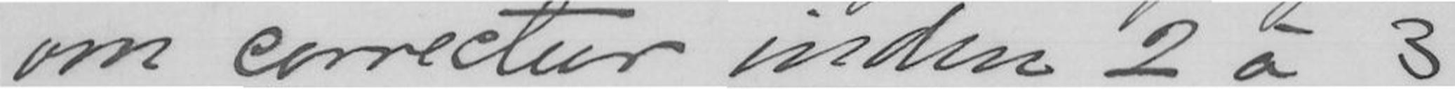om correctur inden 2 à 3
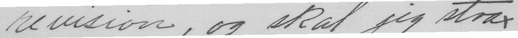revision, og skal jeg strax
